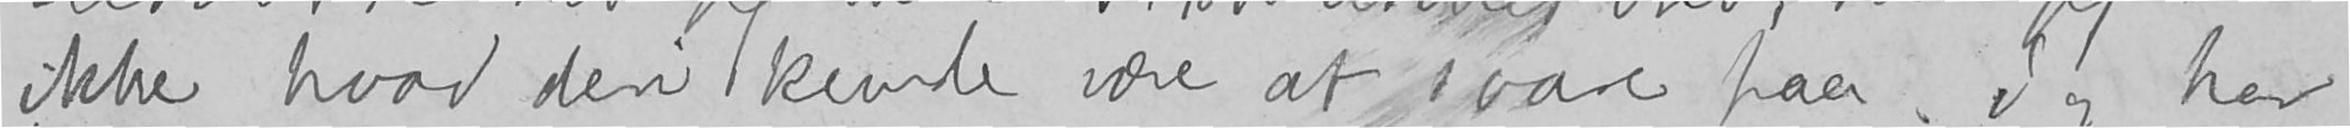ikke hvad der kunde være at svare paa. Jeg har
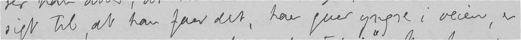sigt til, at han faar det, han gaaer yngre i veien, er
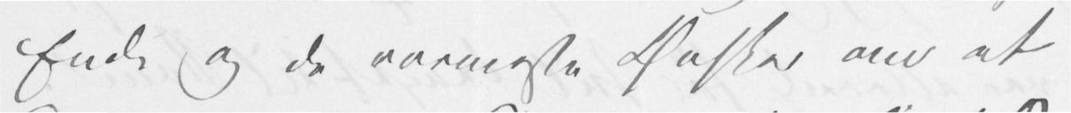Ende og de varmeste Ønsker om at
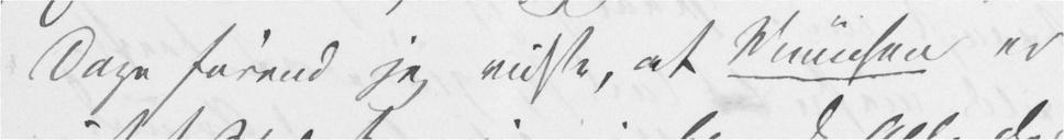Dage førend jeg vidste, at München er
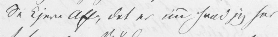Se kjere Alf, det er nu hvad jeg har
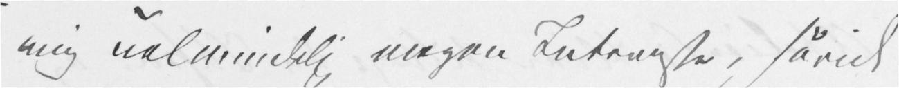mig ualmindelig megen Interesse, såvidt
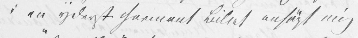i en yderst fornemt Billet ansøgt mig
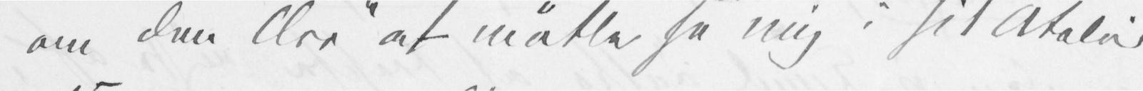om "den Ære" at måtte se mig i sit Atelier
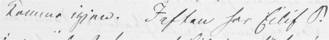komme igjen. Iaften har Eilif P.
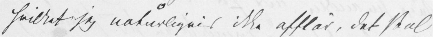hvilket jeg naturligvis ikke afslår, det skal
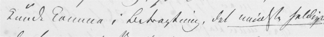kunde komme i Betragtning, det mindste heldige
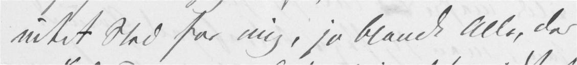intet Sted for mig, jo blandt Alle, der
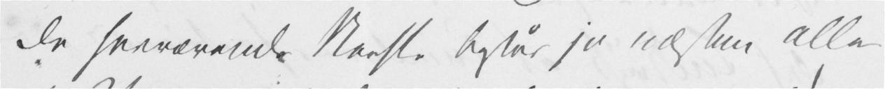De herværende Norske består jo næsten alle
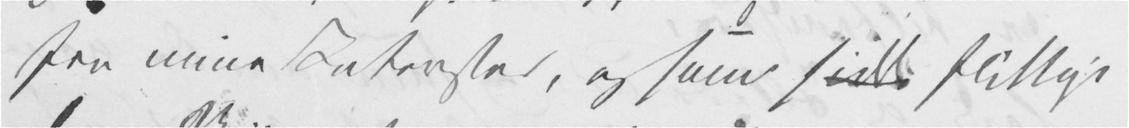fra mine Interesser, og som sidde flittige
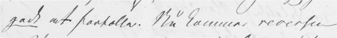godt at fortælle. Nu kommer reversen
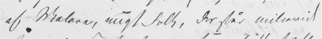af Malere, ungt Folk, der står milevidt
